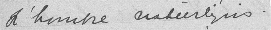L'bonne naturligvis.
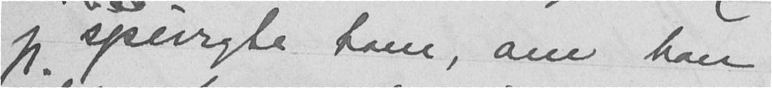jeg spurgte ham, om han
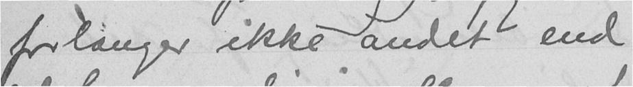forlanger ikke andet end
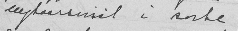nytaarsvisit i sorte
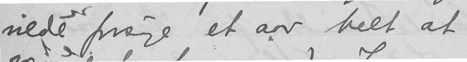vilde forsøge et aar helt at
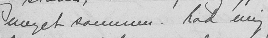meget sammen. bad mig
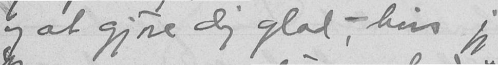og at gjøre dag glad, - hvis jeg
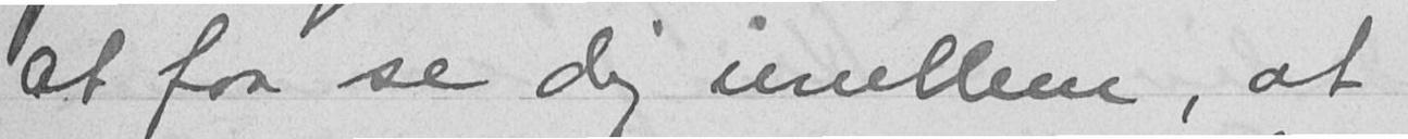at faa se dig imellem, at
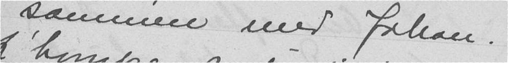sammen med Johan.
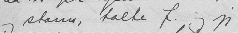og storm, talte J. og jeg
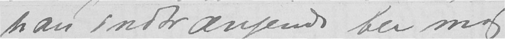han indtrængende ber mig
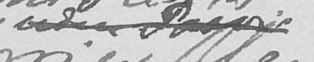uden Papir
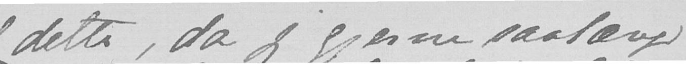dette, da jeg gjerne saalænge
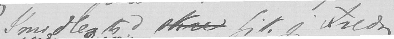Imidlertid skrev fik jeg Fredag
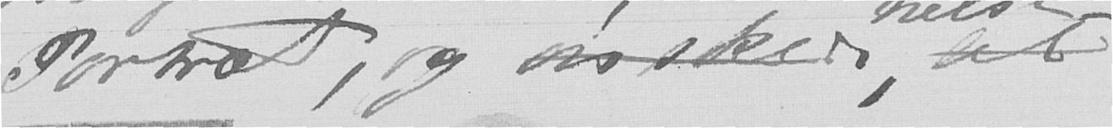Portræt, og ønsker, at
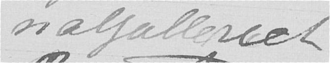nalgalleriet
